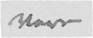vare
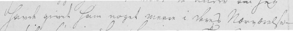havde givet ham noget meere i deres Nærværelse -
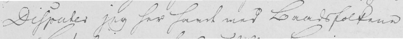Disputer jeg her havde med Baadsfolkene
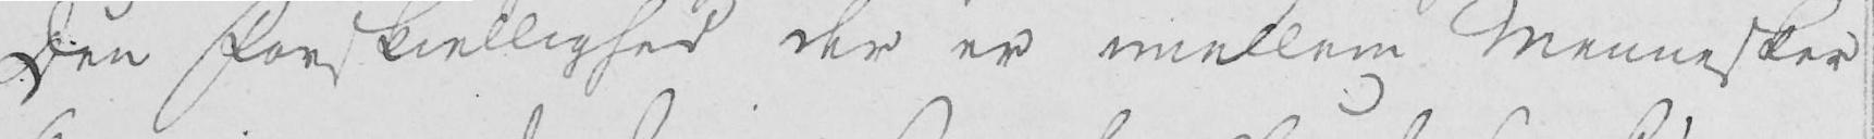Den Forskiellighed der er imellem Mennesker
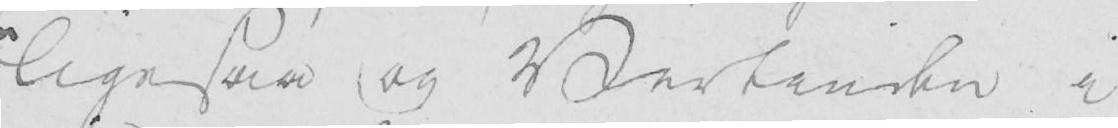ligesaa og Vertinden i
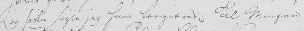og siden søgte jeg ham forgiæves; Til Morgen
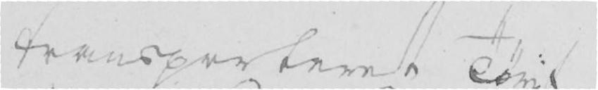transporteret Tøyet
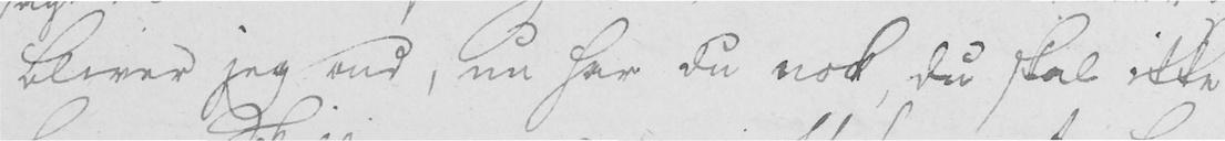bliver jeg ond, nu har du nok, du skal ikke
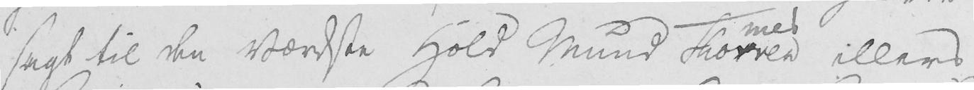sagt til den værste Hold Mund Thormod ellers
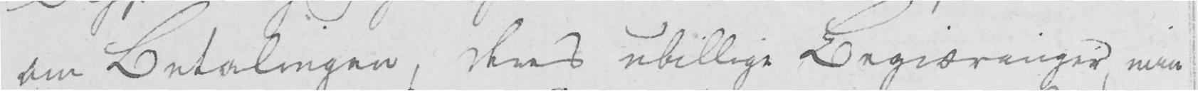om Betalingen, deres ubillige Begiæringer, min
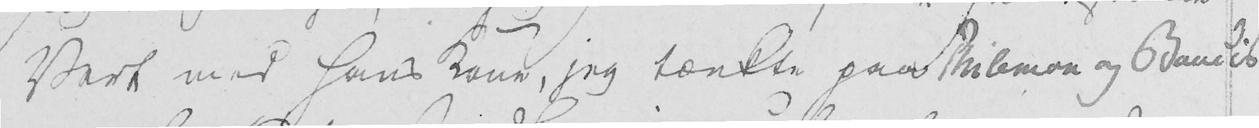Vert med hans Kone, jeg tænkte paa Philemon og Baucis
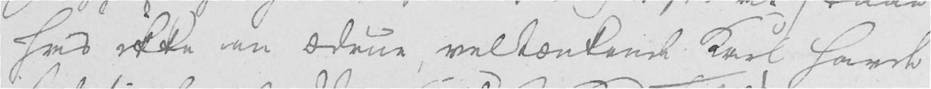hos ikke en ædrue, veltænkende Karl havde
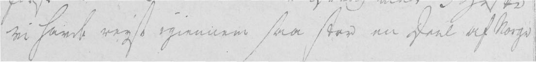vi havde reyst igiennem saa stor en Deel af Norge
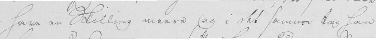have en Skilling meere og i det samme tog han
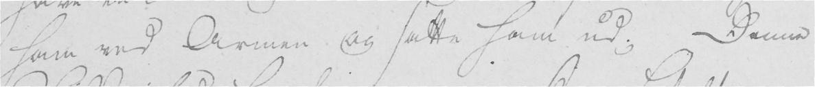ham ved Armen og satte ham ud; Denne
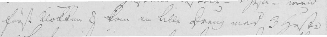først Klokken 8 kom en lille Dreng med 3 Heste
